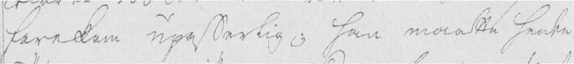forekom upasserlig; han maatte hente
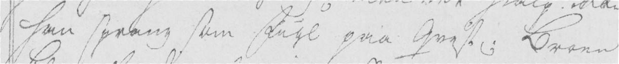han sprang som Fugl paa Qvist; Broen
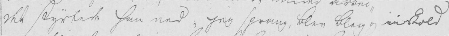det styrtede han ned, jeg sprang, blev bleg og iiskold
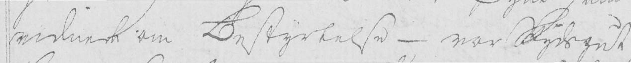vidnede om Bestyrtelse - vor Skydsgut
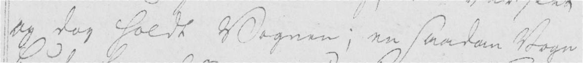og dog holdt Vognen; en saadan Vogn
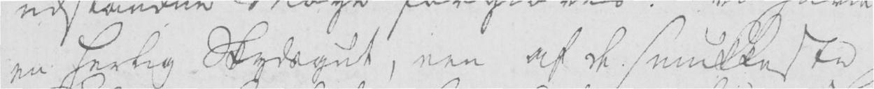en herlig Skydsgut, een af de smukkeste
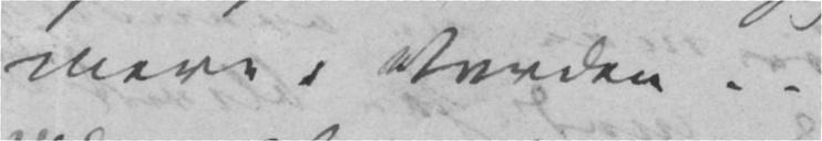mere i Verden – –
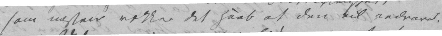som næsten vækker det Haab at den vil vedvare.
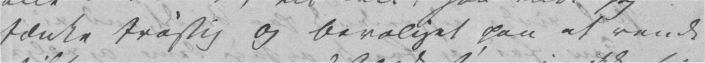tænke trøstig og beroliget paa at vende
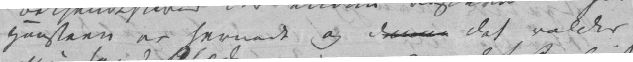Hansteen er hernede og denne det volder
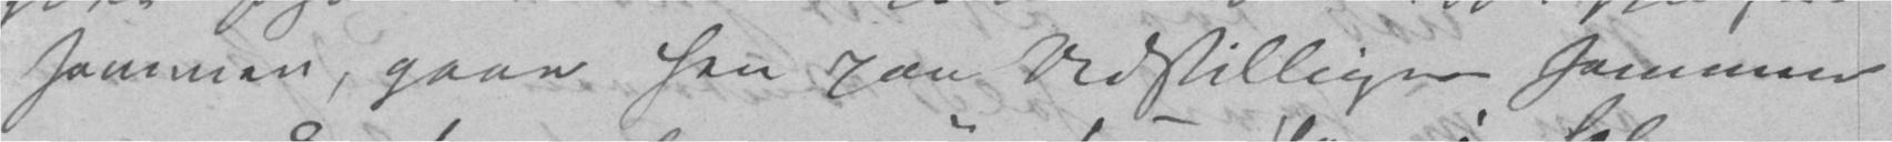sammen, gaae hen paa Udstillingen sammen
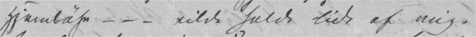Hjemløse – – – vilde holde lidt af mig,
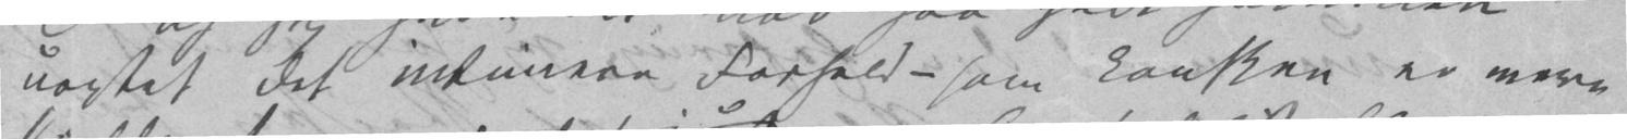uagtet det intimere Forhold – som kanskee er mere
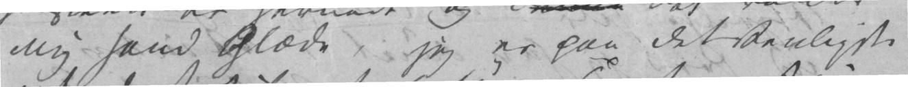mig sand Glæde, jeg er paa det Venligste
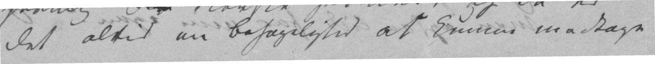det altid en Behagelighed at kunne modtage
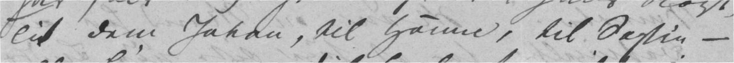til Dem Johan, til Hanne, til Sophie –
